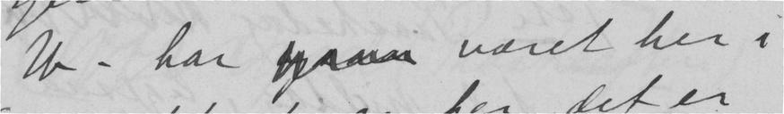W - har  været her i
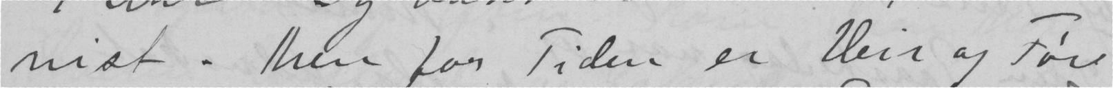vist. Men for Tiden er veir og Føre
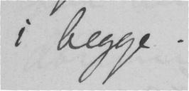i begge.
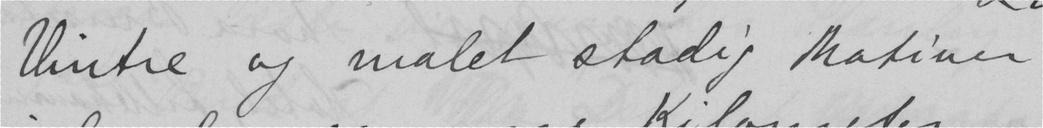Vintre og malet stadig motiver
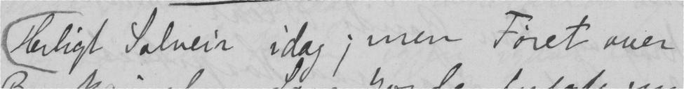Herligt Solveir idag; men Føret over
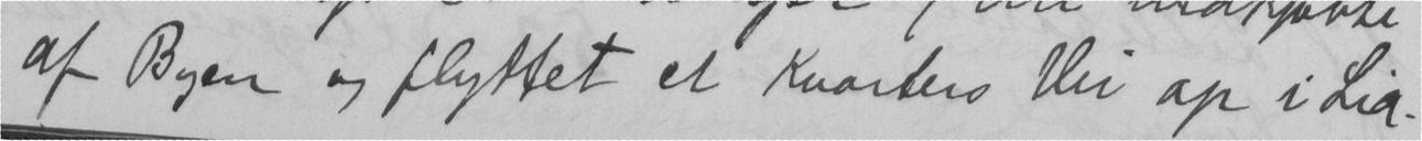af Byen og flyttet et Kvarters vei op i Lia.
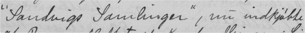"Sandvigs Samlinger", nu indkjøbte
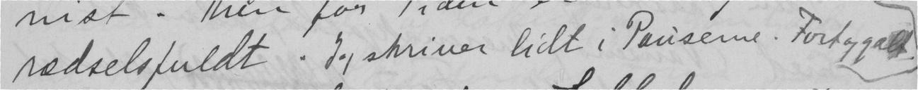rædselsfuldt. Jeg skriver lidt i Pauserne. Fort og galt.
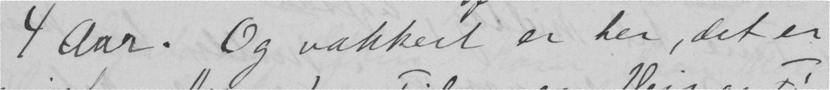4 aar. Og vakkert er her, det er
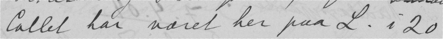Collet har været her paa L. i 20
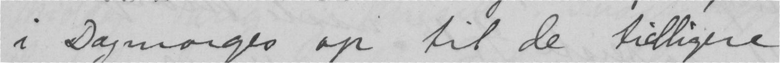i Dagmorges op til de tilligere
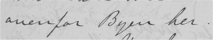ovenfor Byen her.
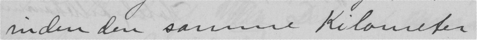inden den samme Kilometer
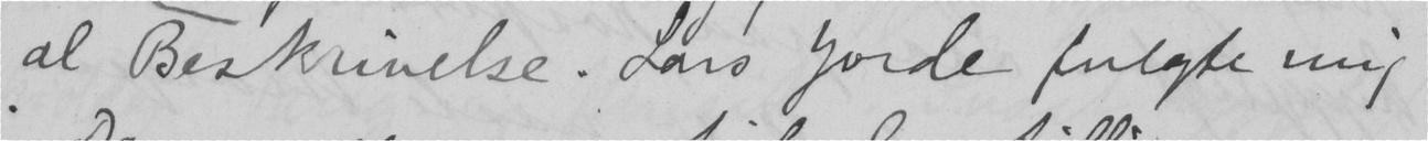al Beskrivelse. Lars jorde fulgte mig
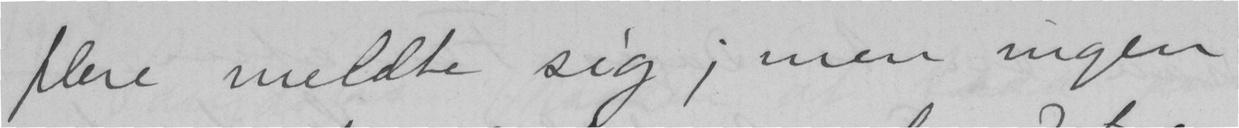flere meldte sig; men ingen
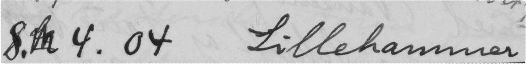8. 4. 04 Lillehammer
